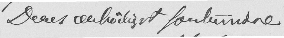Deres ærbødigst forbundne
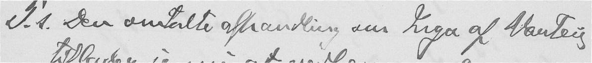P.s. Den omtalte afhandling om Inga af Varteig
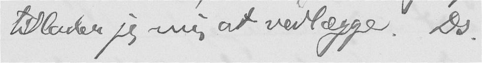tillader jeg mig at vedlægge. Ds.
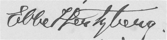Ebbe Hertzberg.
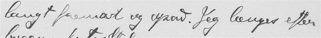langt fremad og opad. Jeg længes efter
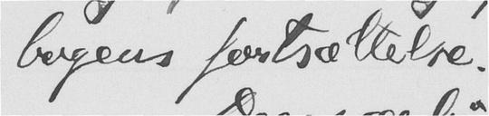bogens fortsættelse.
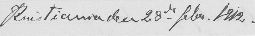Kristiania den 28de febr. 1912.
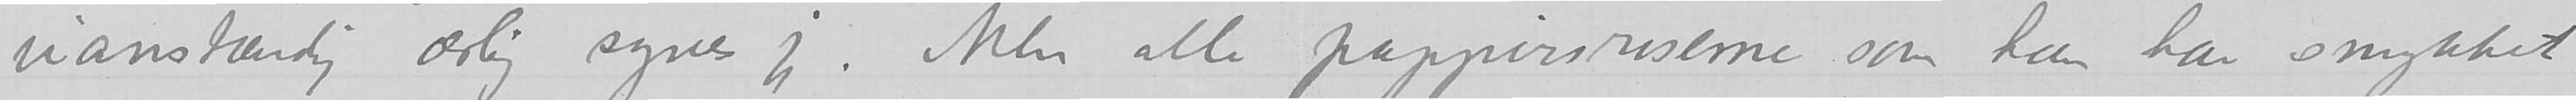uanstændig ærlig synes jeg. Men alle pappirsroserne som han har smykket
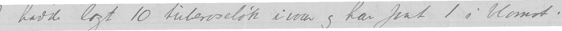Jeg hadde lagt 10 tuberoseløk ivaar og har faat 1 i blomst.
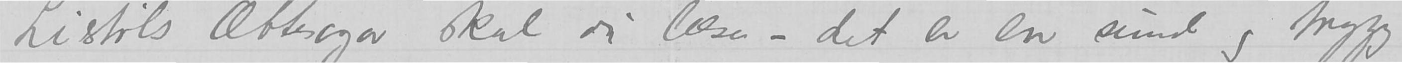Listøls Ættesaga skal du læse – det er en sund og trygg og
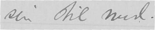sin stil med.
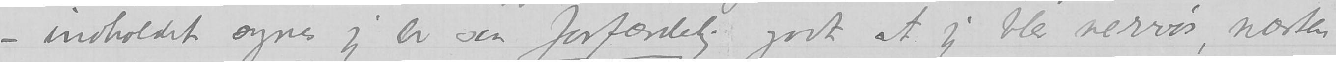indholdet synes jeg er saa forfærdelig godt at jeg blev nervøs, næsten
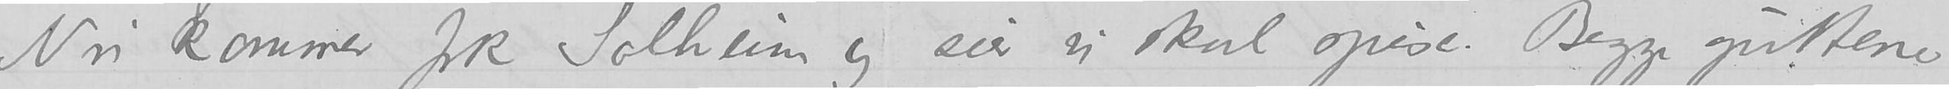Nu kommer frk Solheim og sier vi skal spise. Begge guttene
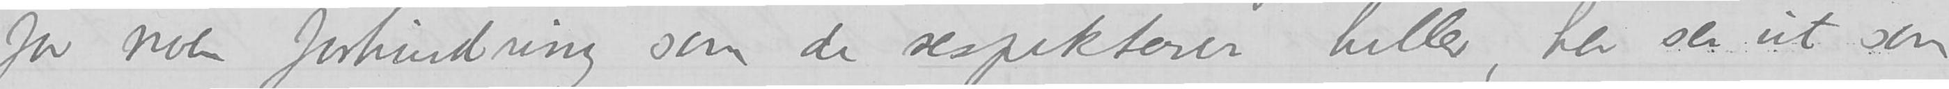for noen forhindring som de respekterer heller, her ser ut som
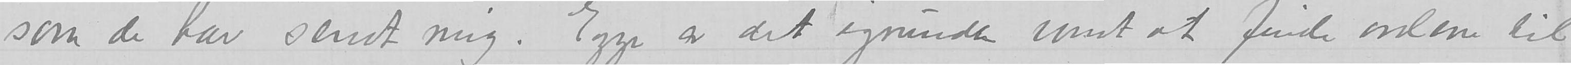som de har sendt mig. Egge er det igrunden nemdt at finde ordene til –
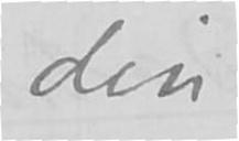din
